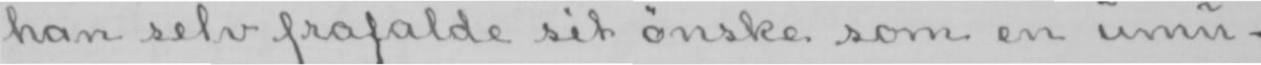han selv frafalde sit ønske som en umu-
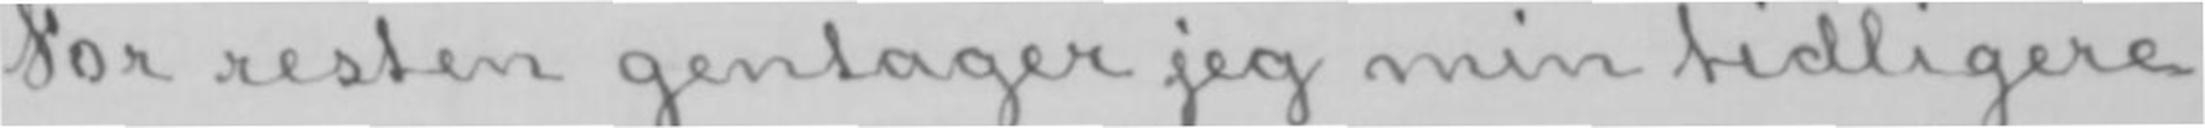For resten gentager jeg min tidligere
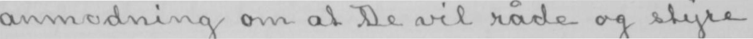anmodning om at De vil råde og styre
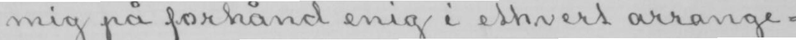mig på forhånd enig i ethvert arrange-
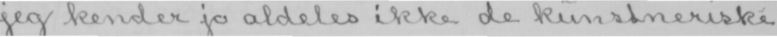jeg kender jo aldeles ikke de kunstneriske
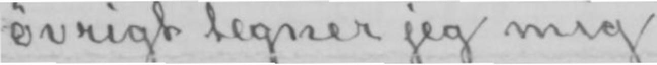øvrigt tegner jeg mig
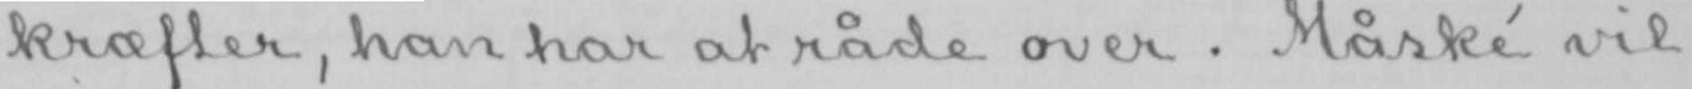kræfter, han har at råde over. Måské vil
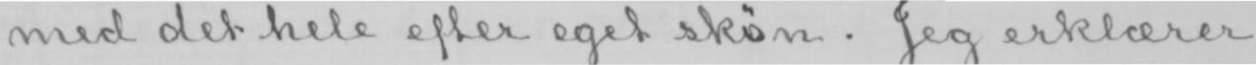med det hele efter eget skøn. Jeg erklærer
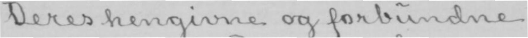Deres hengivne og forbundne
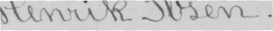Henrik Ibsen.
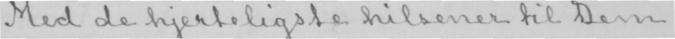Med de hjerteligste hilsener til Dem
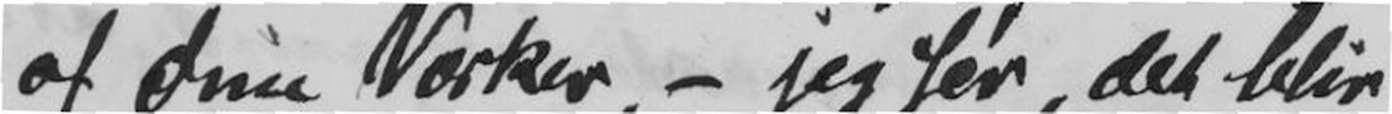af dine Værker, - jeg ser, det blir
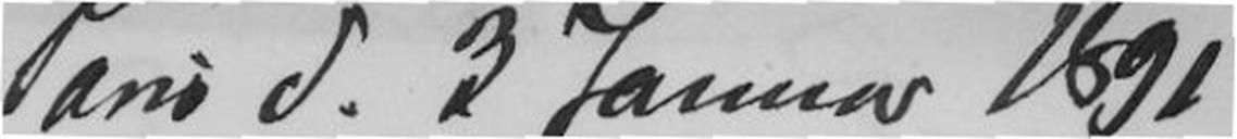Paris d. 3 Januar 1891
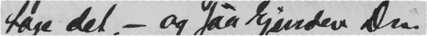tage det, - og saa kjender Du
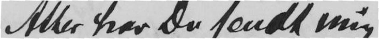Atter har Du sendt mig
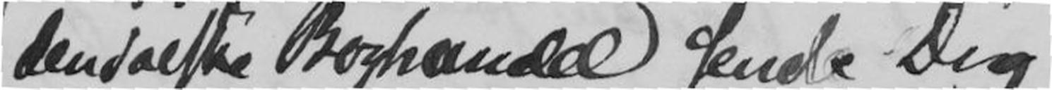dendalske Boghandel sende Dig
